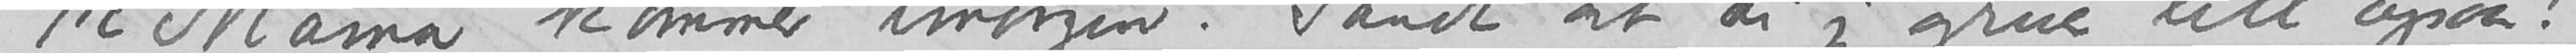20/12 Mama kommer imorgen. Sandt at si jeg gruer litt ogsaa!
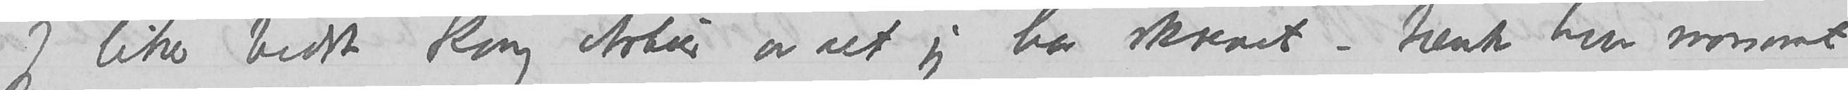Jeg liker bedst kong Arthur av alt jeg har skrevet – tænk hvor morsomt
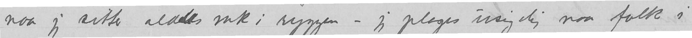naar jeg sitter aldeles rak i ryggen – jeg plages usigelig naar folk i
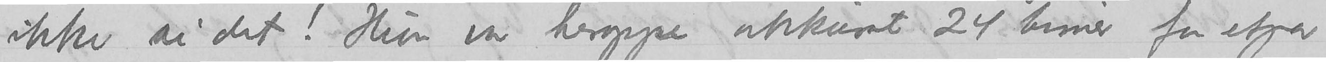ikke si det! Hun var heroppe akkurat 24 timer for etpar
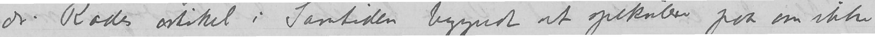dr. Rodes artikel i Samtiden begyndt at spekulere paa om ikke
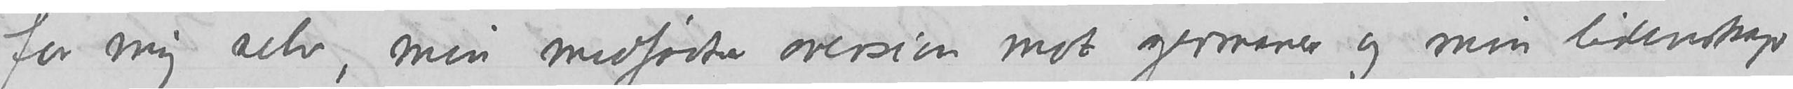for mig selv, min medfødte aversion mot germaner og min lidenskap
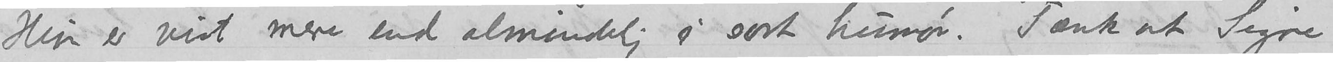Hun er vist mere end almindelig i sort humør. Tænk at Signe
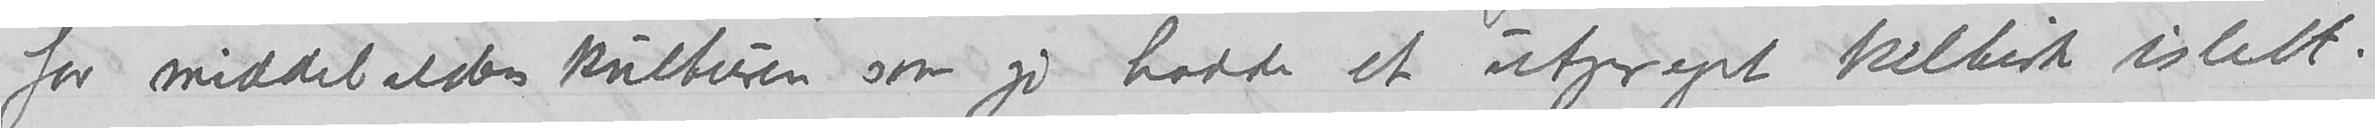for middelalderskulturen som jo hadde et utpreget keltisk islett.
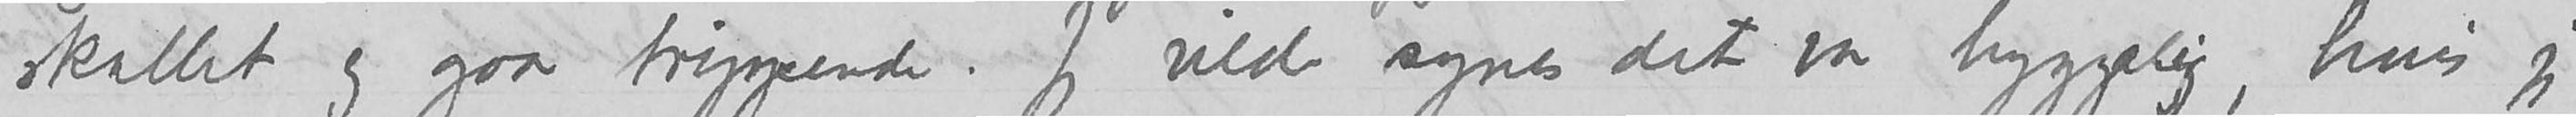skallet og gaar trippende. Jeg vilde synes det var hyggelig, hvis jeg
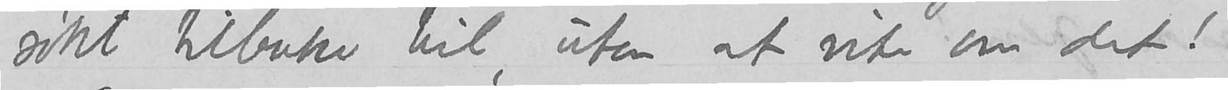søkt tilbake til, uten at vite om det!
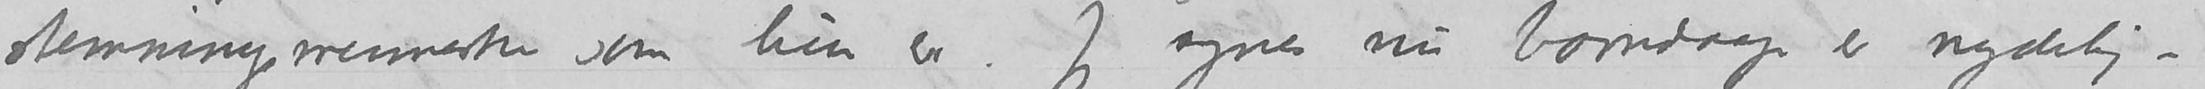stemningsmenneske som hun er. Jeg synes nu barndaap er nydelig –
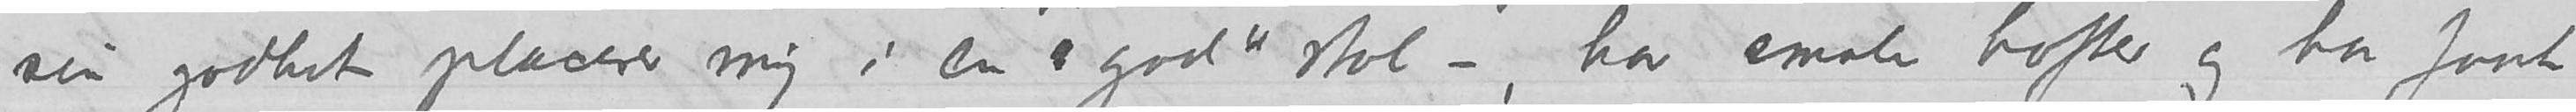sin godhet placerer mig i en «god» stol –, har smale hofter og har faat
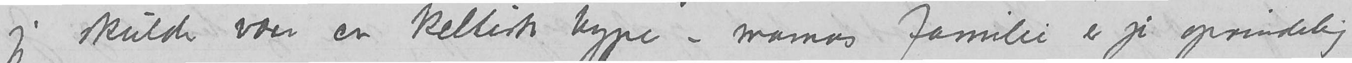jeg skulde være en keltisk type – mamas familie er jo oprindelig
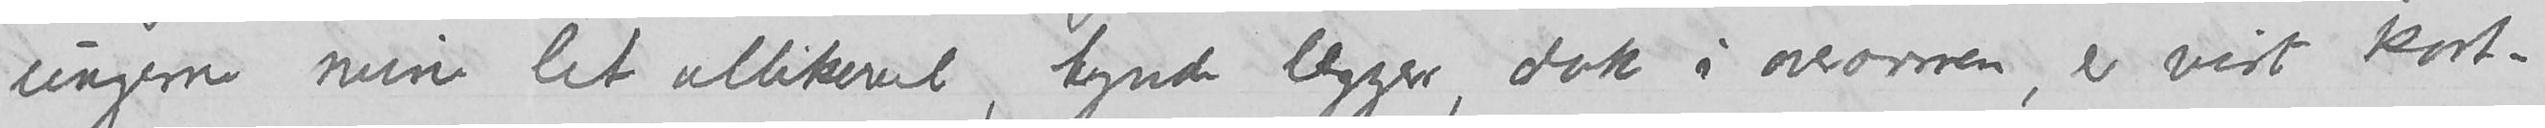ungerne mine let allikevel, tynde lægger, stærk i overarmen, er vist kort-
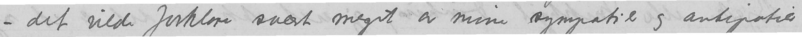det vilde forklare svært meget av mine sympatier og antipatier
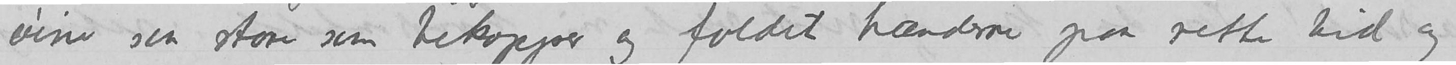øine saa store som tekopper og foldet hænderne paa rette tid og
